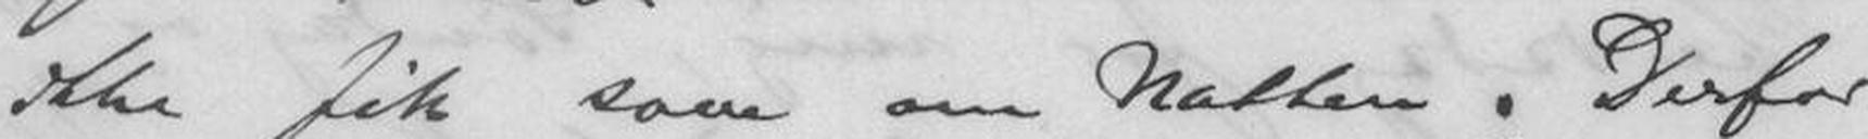ikke fik sove om Natten. Derfor
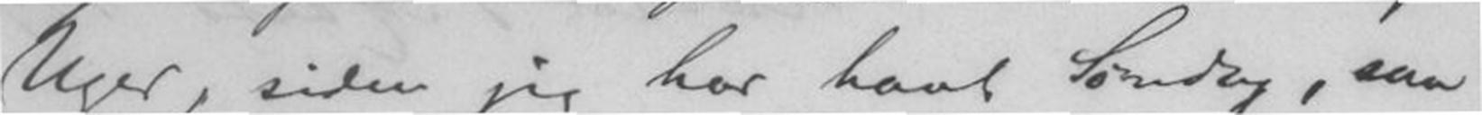Uger, siden jeg har havt Søndag, saa
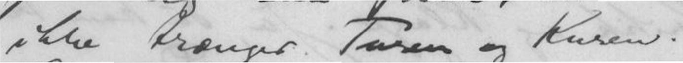ikke trænger Turen og Kuren. -
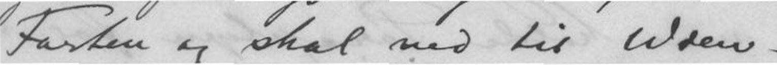Farten og skal ned til Wien.
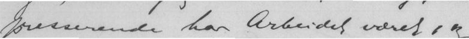presserende har Arbeidet været, og
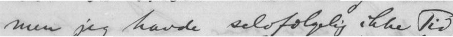men jeg havde selvfølgelig ikke Tid
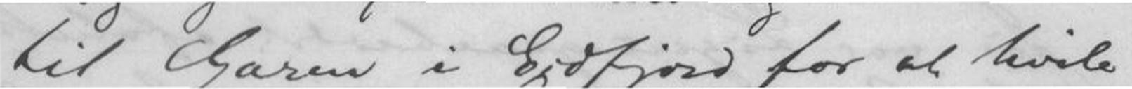til Garen i Eidfjord for at hvile
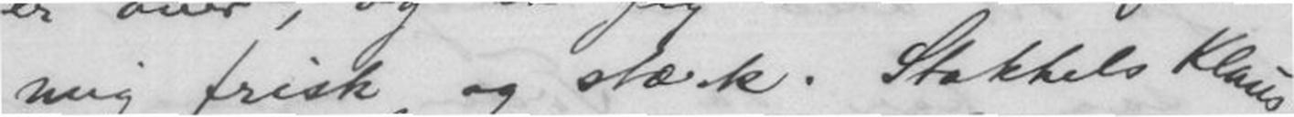mig frisk og stærk. Stakkels Klaus
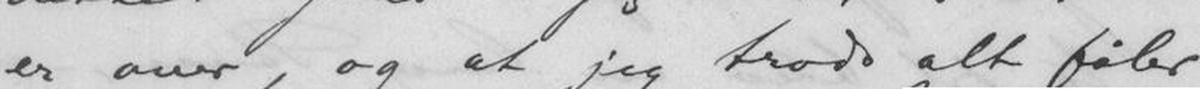er over, og at jeg trods alt føler
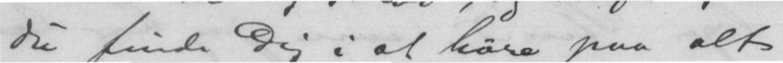du finde Dig i at høre paa alt
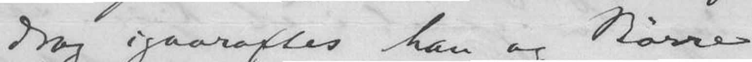drog igaaraftes han og Børre
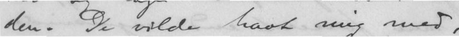den. De vilde havt mig med,
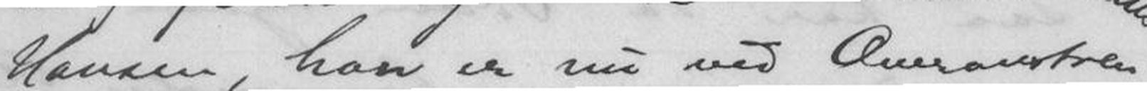Hansen, han er nu ved Overanstren-
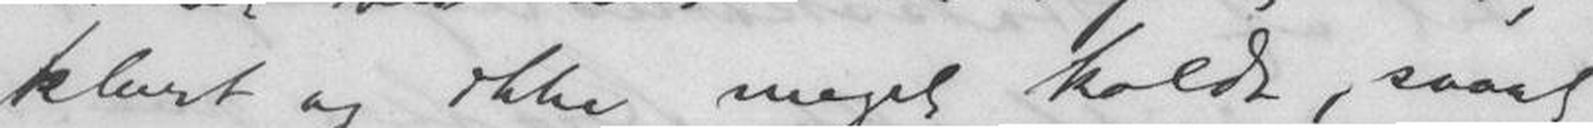klart og ikke meget koldt, saaat
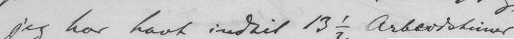jeg har havt indtil 13 ½ Arbeidstimer
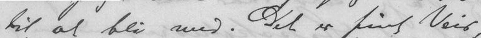til at bli med. Det er fint Veir,
